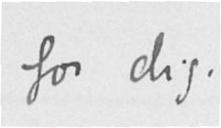for dig.
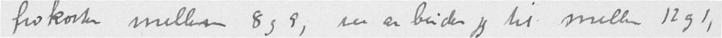frokosten mellem 8 og 9, saa arbeider jeg til mellem 12 og 1,
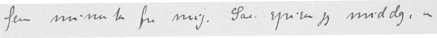fem minuter fra mig. Saa spiser jeg middag, en
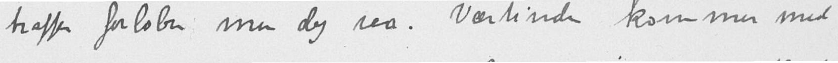træffer forløber min dag saa. Værtinden kommer med
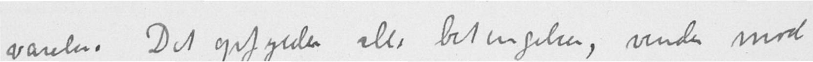værelse. Det opfylder alle betingelser, vender mod
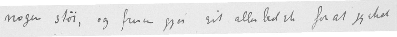nogen støi, og fruen gjør sit aller bedste for at jeg skal
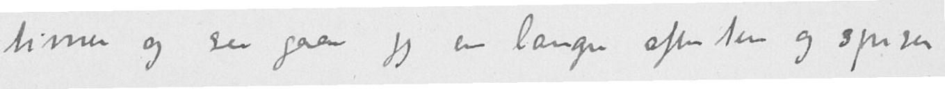timer og saa gaar jeg en længre aftentur og spiser
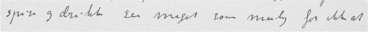spise og drikke saa meget som mulig for ikke at
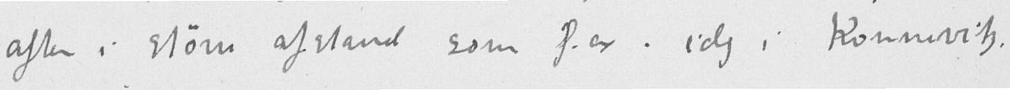aften i større afstand som f.ex. i dag i Konnevitz.
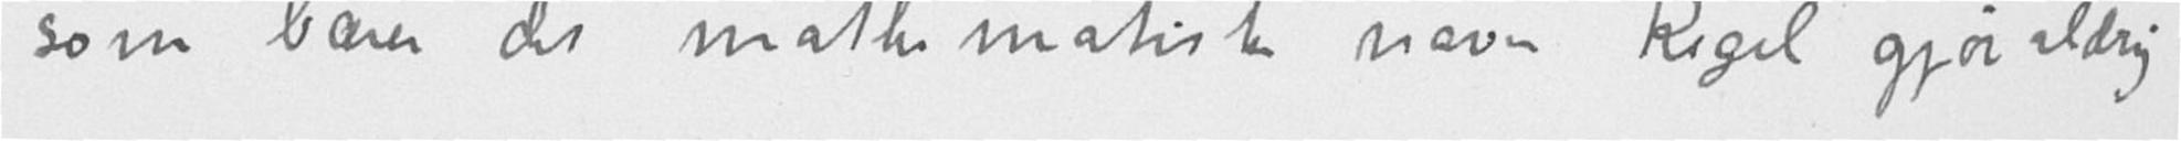som bærer det mathematiske navn Kegel gjør aldig
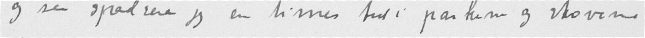og saa spadserer jeg en times tid i parken og skovene
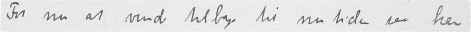For nu at vende tilbage til nutiden saa har
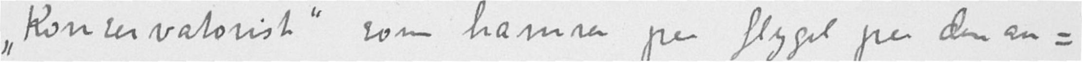"Konservatorist" som hamrer paa flygel paa den an-
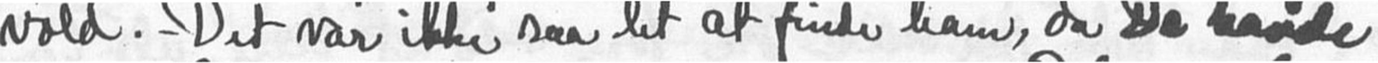vold. - Det var ikke saa let at finde ham, da De havde
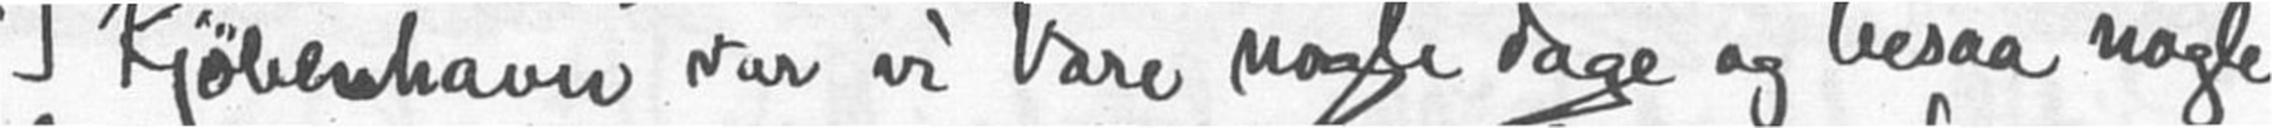I Kjøbenhavn var vi bare nogle dage og besaa nogle
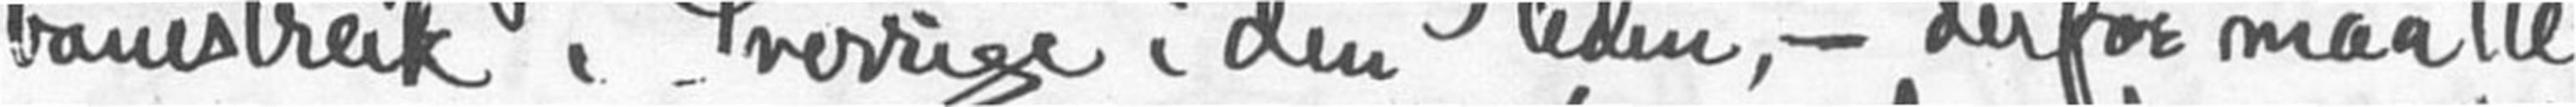banestreik i Sverrige i den tiden, - derfor maatte
